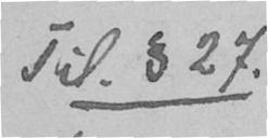Til § 27.
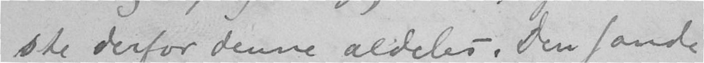ste derfor denne aldeles. Den sande
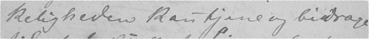keligheden kan tjene og bidrage
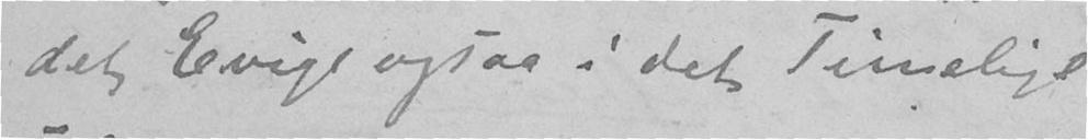det Evige ogsaa i det Timelige
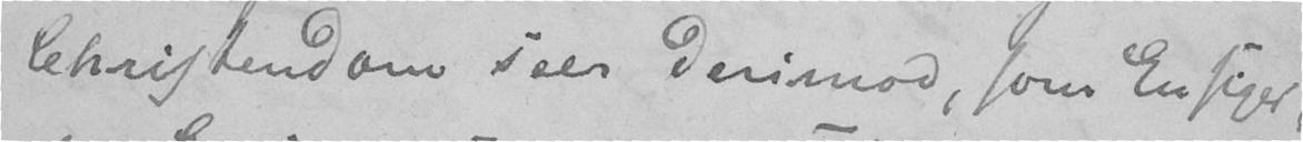Christendom seer derimod, som En siger,
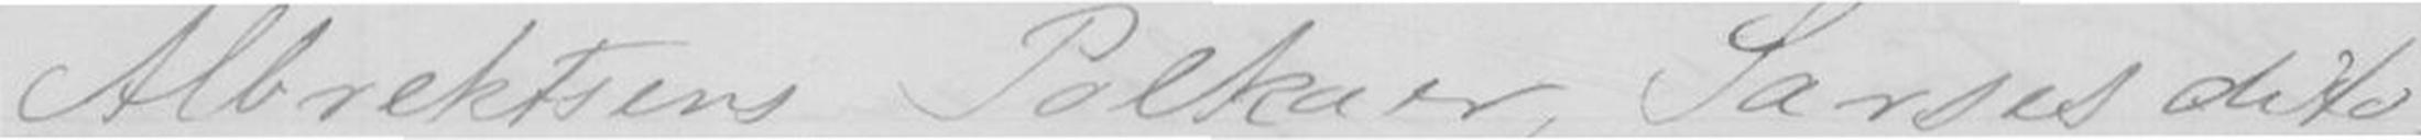Albrektsens Polkaer, Sarses dito
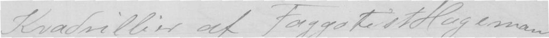Kvadrillier af Faggotist Hageman
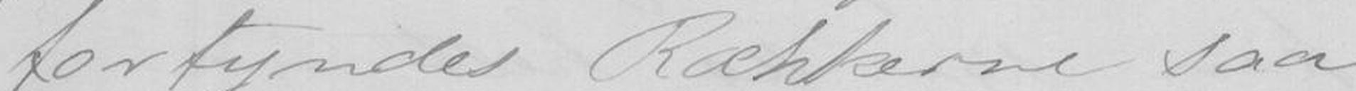fortyndes Rækkerne saa
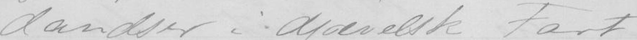dandser i djævelsk Fart
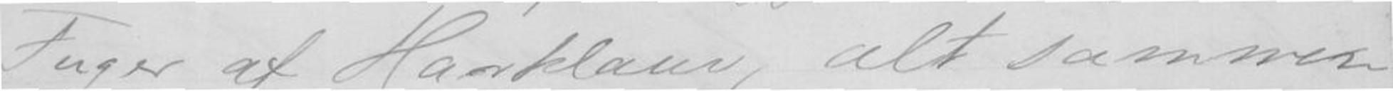Fuger af Haarklauv, alt sammen
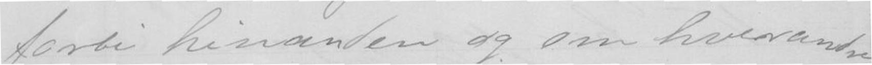forbi hinanden og om hverandre,
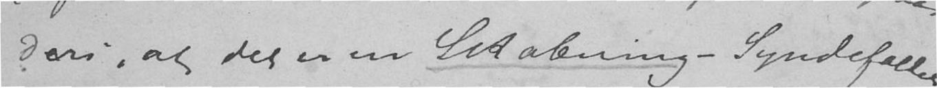deri, at det er en Skabning –Syndefallet
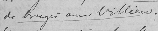de bruges om Villien.
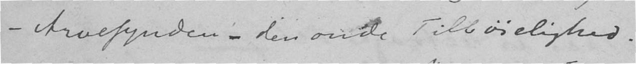– Arvesynden – den onde Tilbøielighed.
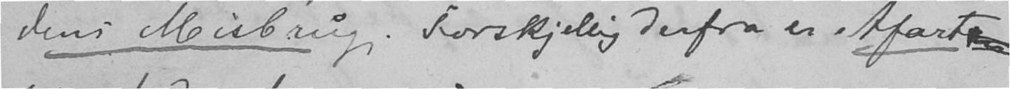dens Misbrug. Forskjellig derfra er Afart-
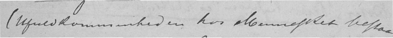(Ufuldkommenheden hos Mennesket bestaaer
